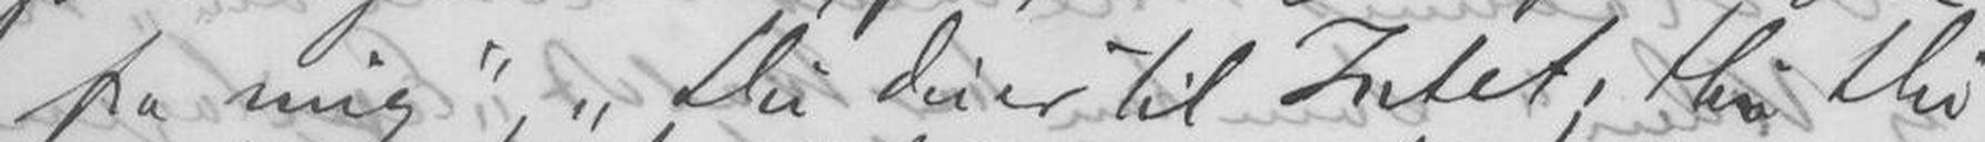fra mig, Du duer til Intet; thi Du
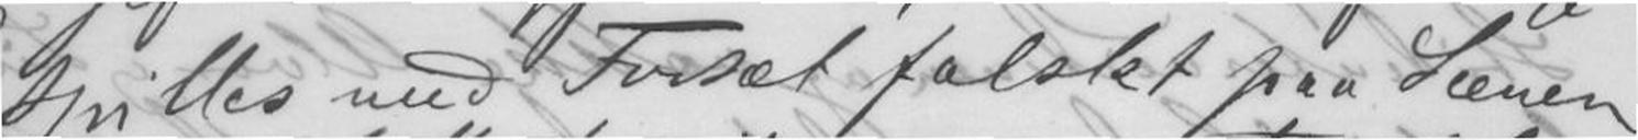spilles med Forsæt falskt paa Scenen
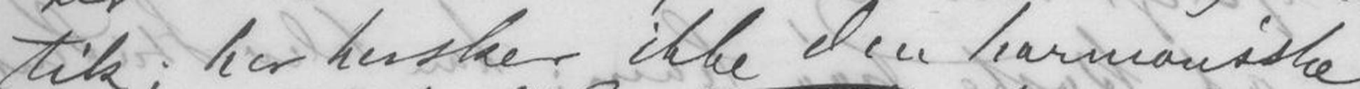tik; her hersker ikke den harmoniske
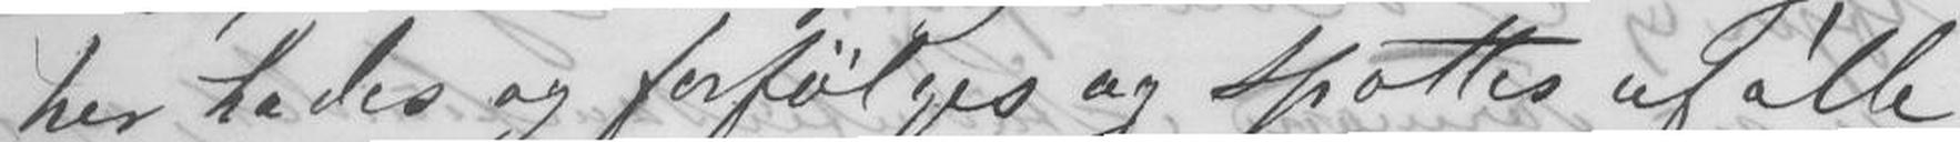her hades og forfølges og spottes af alle
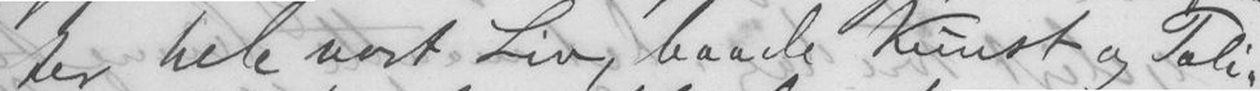ter hele vort Liv, baade Kunst og Poli-
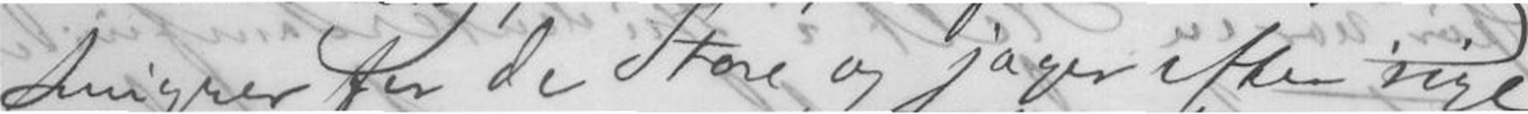smigrer for de Store og jager efter rige
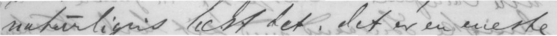naturligvis læst det, det er en eneste
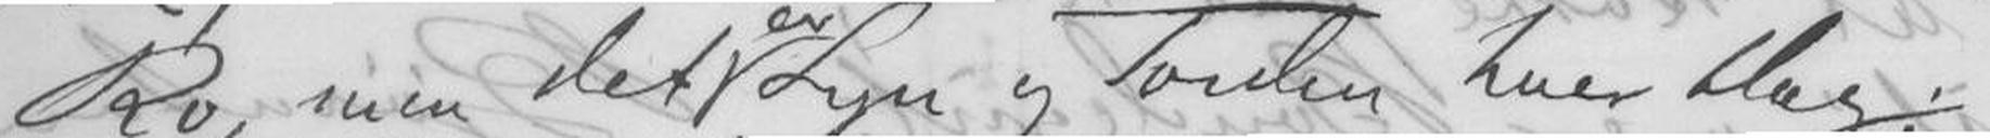Ro, men det er Lyn og Torden hver Dag;
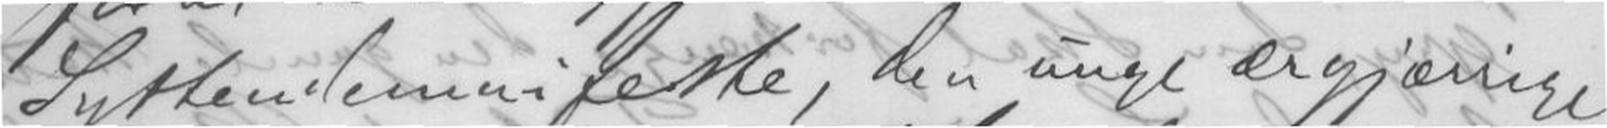Syttendemaifester, den unge ærgjærrige
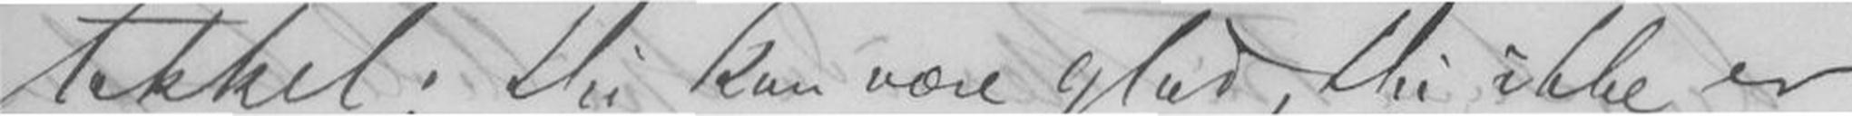takkel; Du kan være glad, Du ikke er
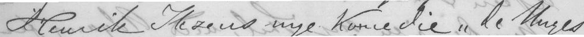Henrik Ibsens nye Komedie de Unges
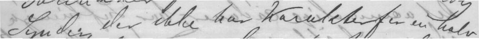Synder, der ikke har Karakter for en halv
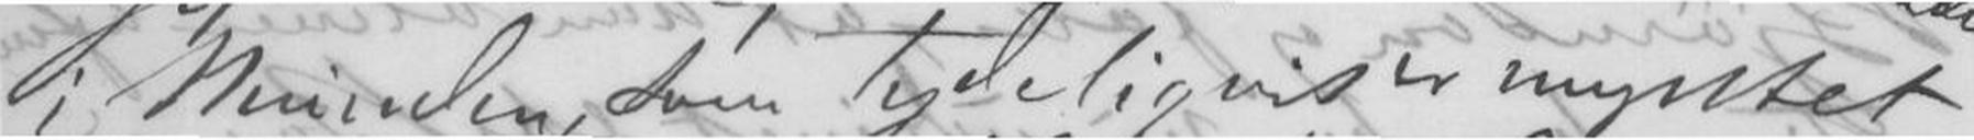i Munden, som tydeligvis er myntet
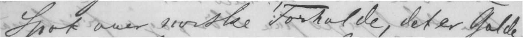Spot over norske Forholde, det er Galde
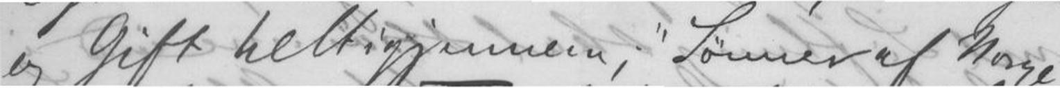og Gift helt igjennem; "Sønner af Norge"
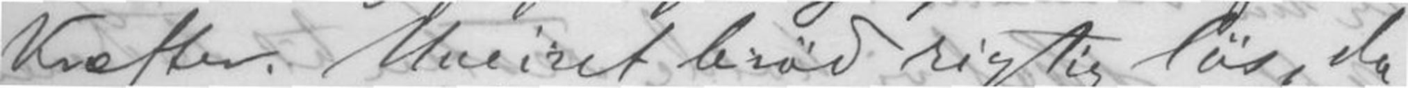Kræfter. Uveiret brød rigtig løs, da
